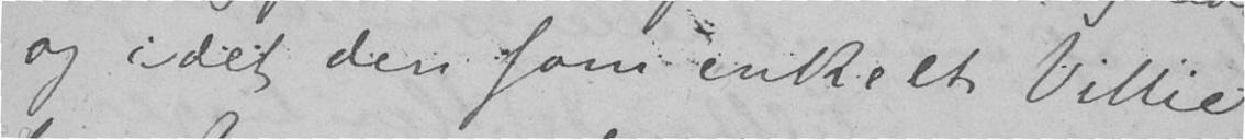og idet den som enkelt Villie
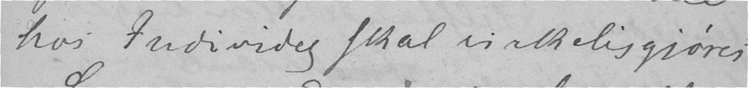hos Individet skal virkeliggjøres
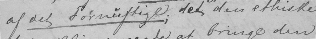af det Fornuftige; det den ethiske
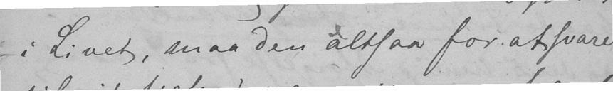i Livet, maa den altsaa for at svare
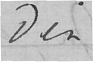Din
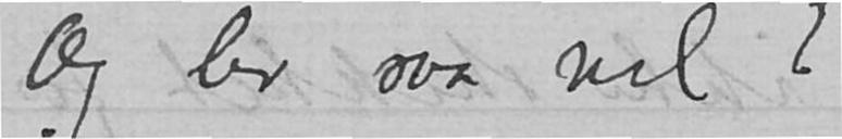Og lev saa vel!
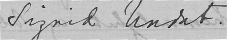Sigrid Undset.
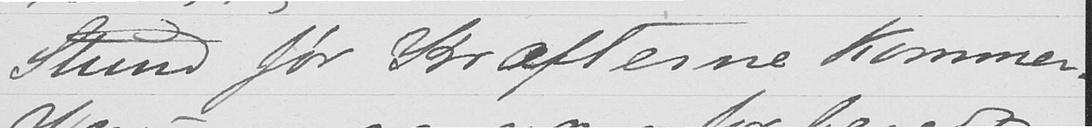Stund før Kræfterne kommer.
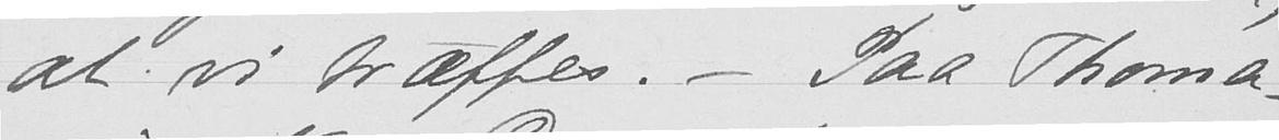at vi træffes. - Paa Thoma-
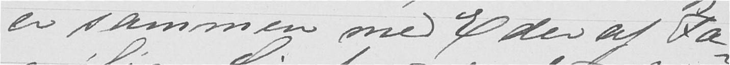er sammen med Eder af Fa-
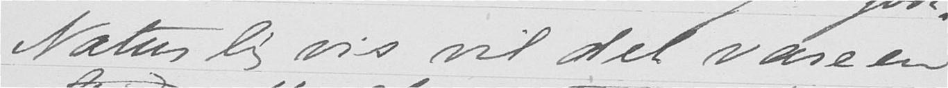Naturligvis vil det vare en
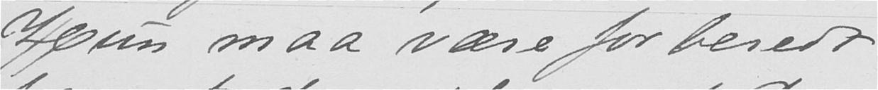Hun maa være forberedt
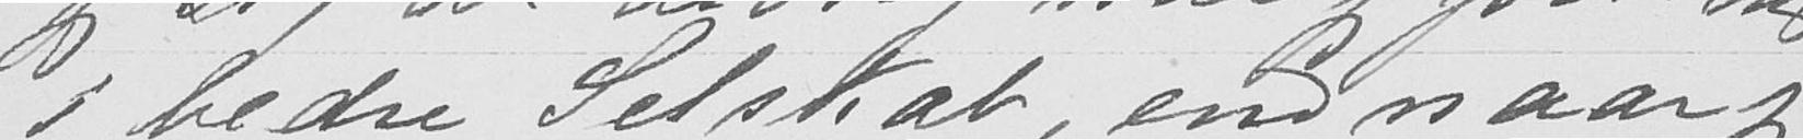i bedre Selskab end naar jeg
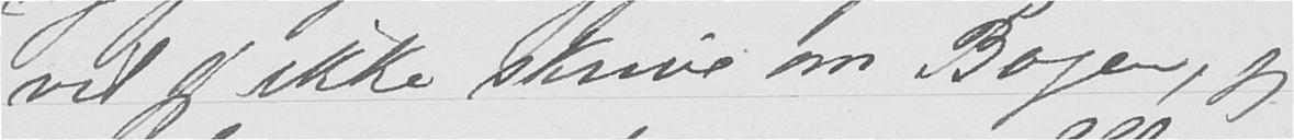vil jeg ikke skrive om Bogen, jeg
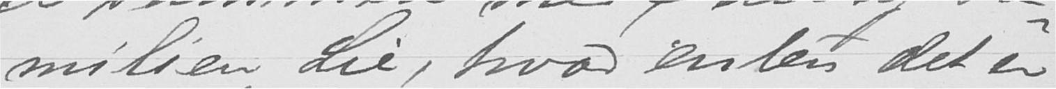milien Lie, hvad enten det er
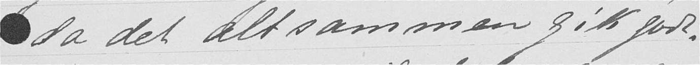da det alt sammen gik godt.
